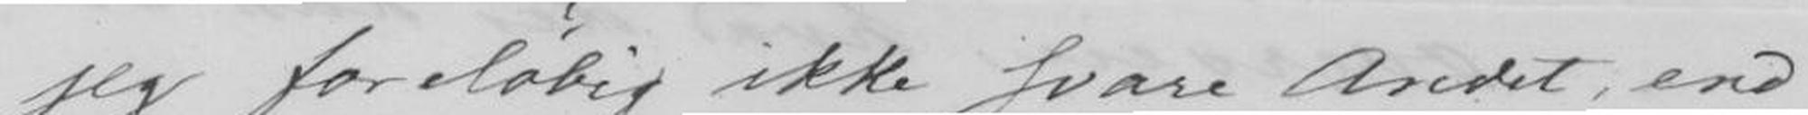jeg foreløbig ikke svare Andet, end
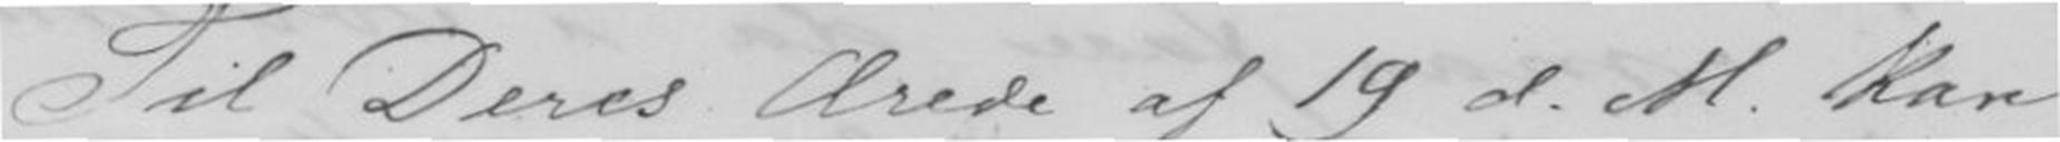Til Deres Ærede af 19 d. M. Kan
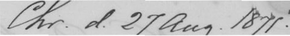Chr. d. 27 Aug. 1871.
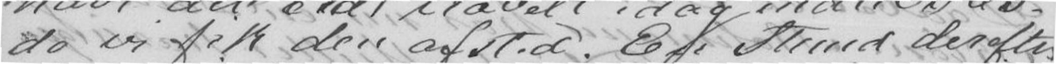da vi fik den afsted. En Stund derefter
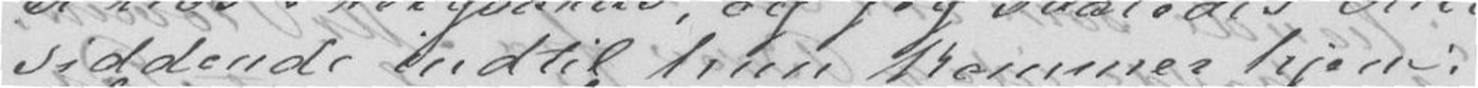siddende indtil hun kommer hjem.
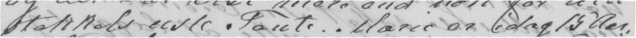stakkels usle Tante. Marie er idag 15 Aar,
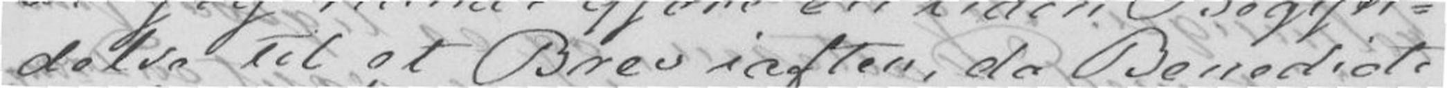delse til et Brev iaften, da Benedicte
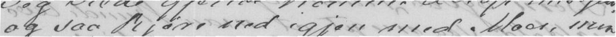og saa kjøre ned igjen med Moer, men
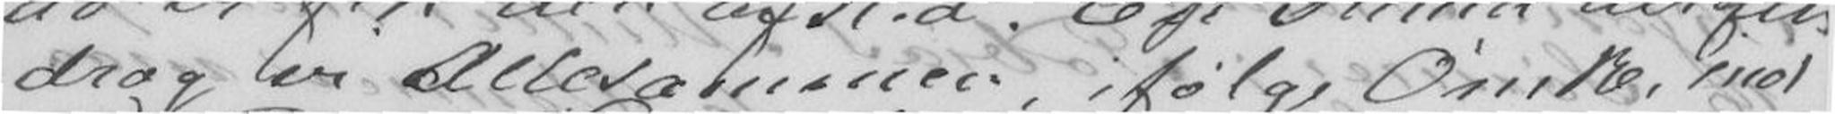drog vi Allesammen, ifølge Ønske ind
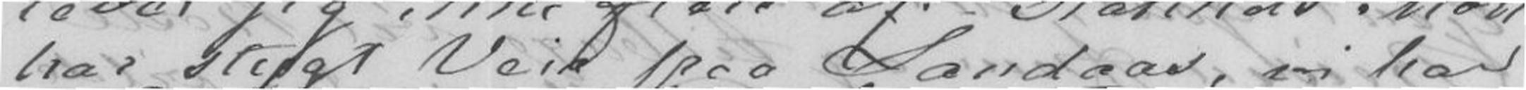har stygt Veir paa Landaas, vi har
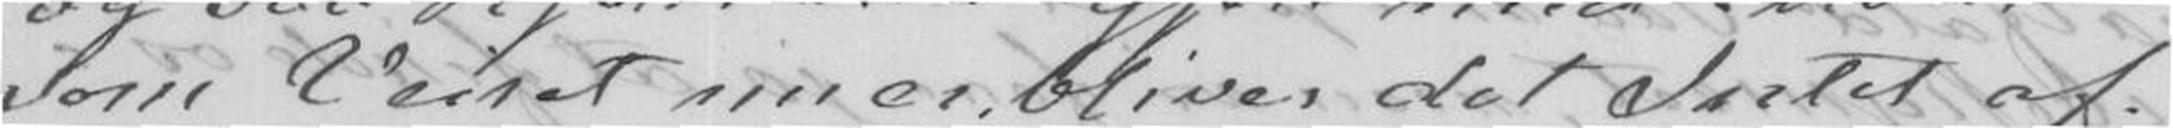som Veiret nu er, bliver det Intet af.
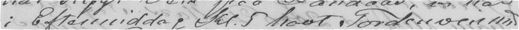i Eftermiddag Kl.5 havt Tordenveir med
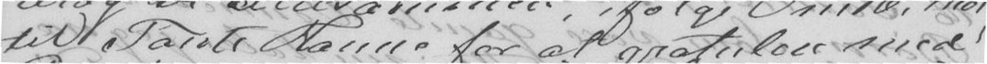til Tante Hanne for at gratulere med
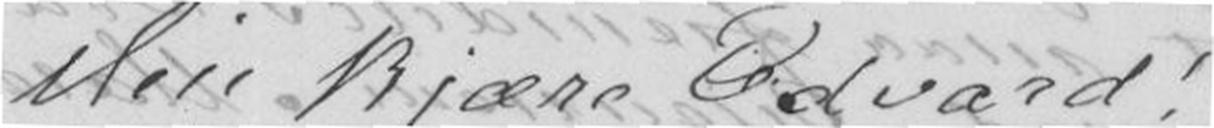Min kjære Edvard!
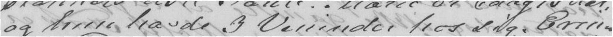og hun havde 3 Veninder hos sig. Erin-
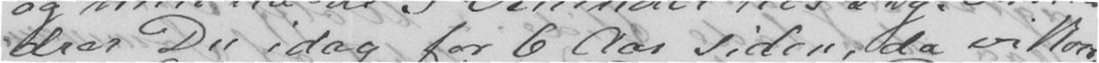drer Du idag for 6 Aar siden, da vi kom
